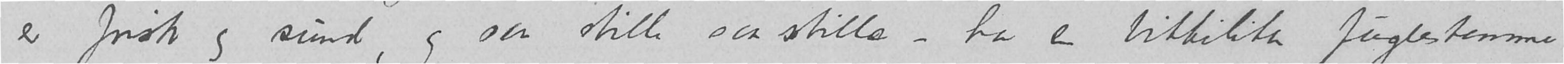er frisk og sund, og saa stille saa stille – har en bitteliten fuglestemme
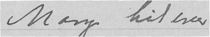Mange hilsener
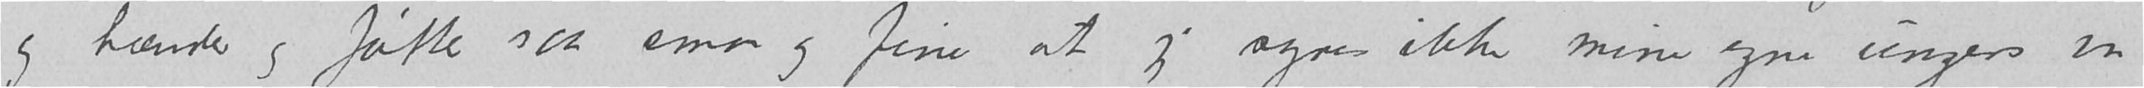og hænder og føtter saa smaa og fine at jeg synes ikke mine egne ungers var
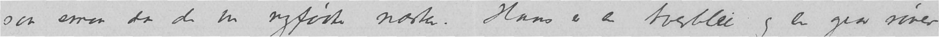saa smaa da de var nyfødte næsten.  Hans er en <tverblu> og en <egen> røver
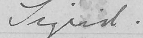Sigrid.
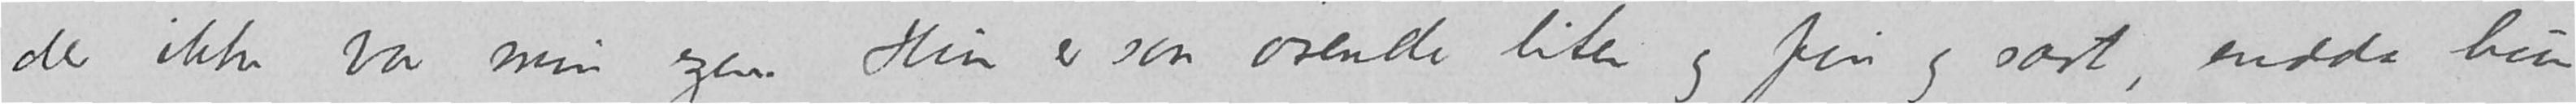der ikke var min egen.  Hun er saa ørende liten og fin og sart, endda hun
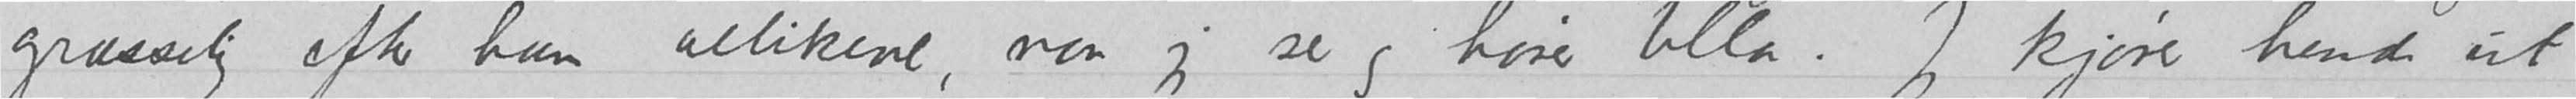græsselig efter ham allikevel, naar jeg ser og hører Ulla.  Jeg kjører hende ut
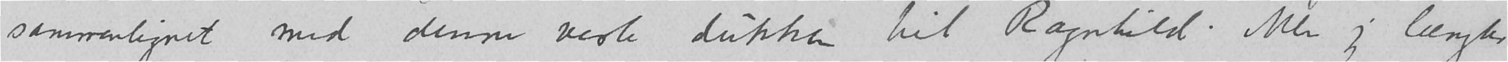sammenlignet med denne vesle dukken til Ragnhild.  Men jeg længter
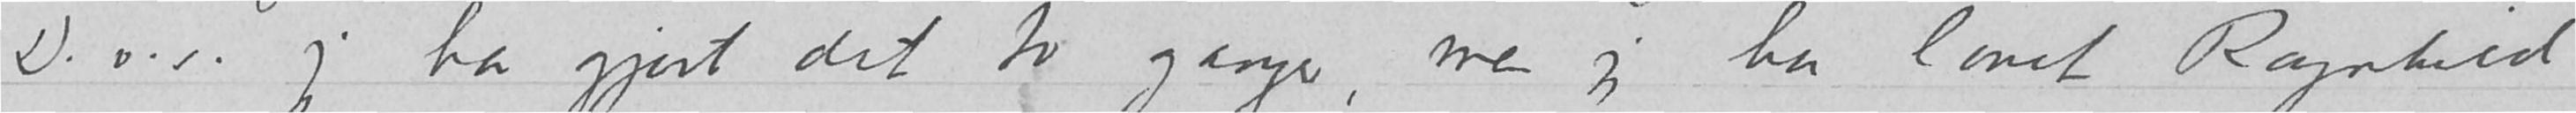D.v.s. jeg har gjort det to ganger, men jeg har lovet Ragnhild
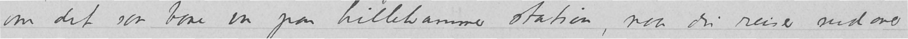om det saa bare var paa Lillehammer station, naar du reiser nedover
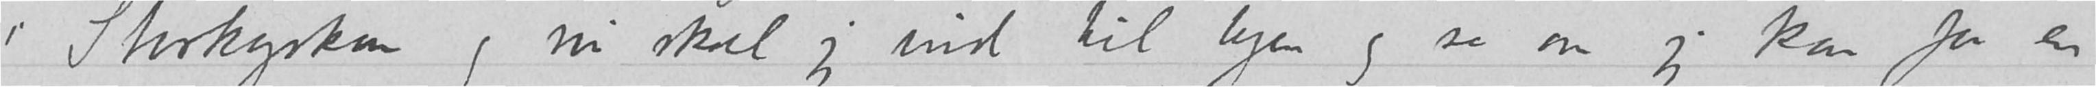i Storkyrkan og nu skal jeg ind til byen og se om jeg kan faa en
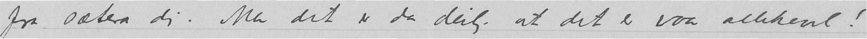fra sætera di.  Men det er da deilig at det er vaar allikevel!
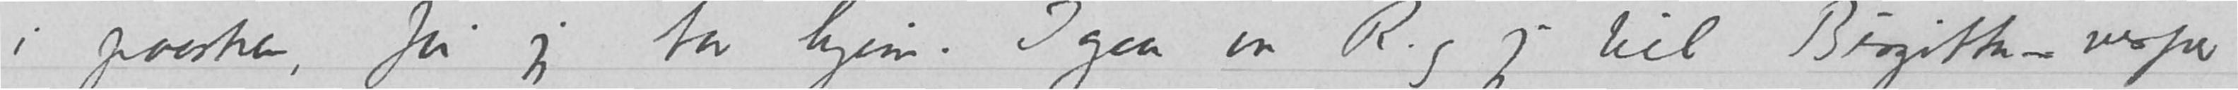i paasken, før jeg tar hjem.  Igaar var R. og jeg til Birgitta-vesper
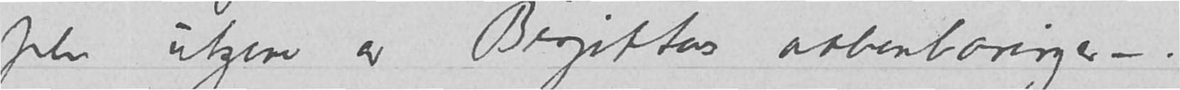pen utgave av Birgittas aabenbaringer –.
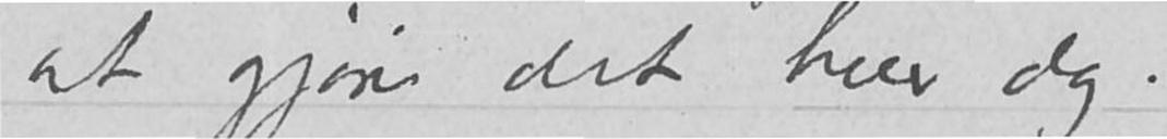at gjøre det hver dag.
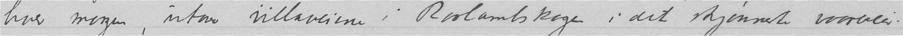hver morgen, utover villaveiene i Raalambskogen i det skjønneste vaarveir.
